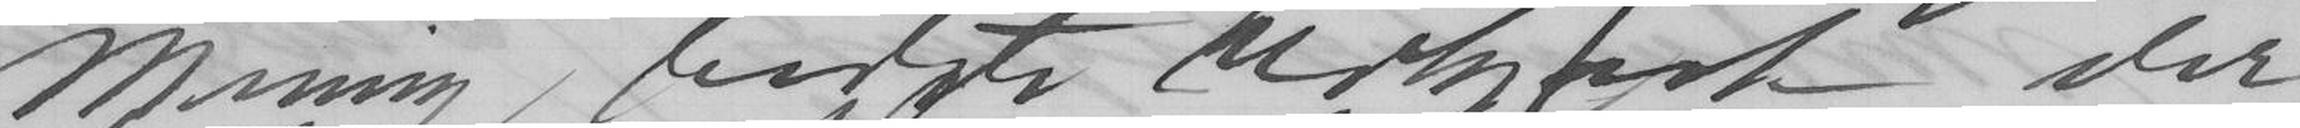Mening bedste Udkast der
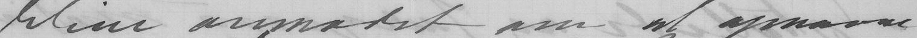blive anmodet om at opnævne
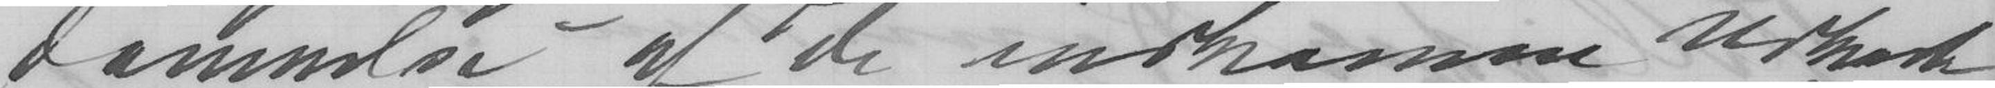dømmelse - af de indkomne Udkast
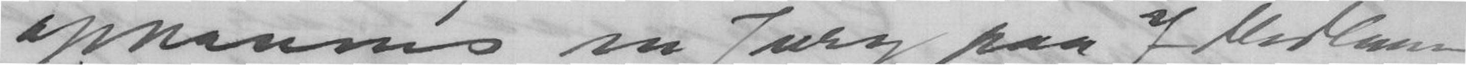opnævnes en Jury paa 7 Medlemmer.
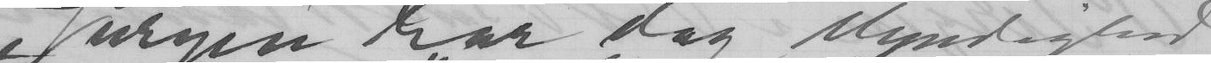Juryen har dog Myndighed
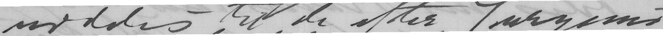uddeles til de efter Juryens
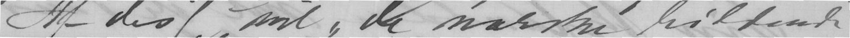Af disse vil "de norske bildende
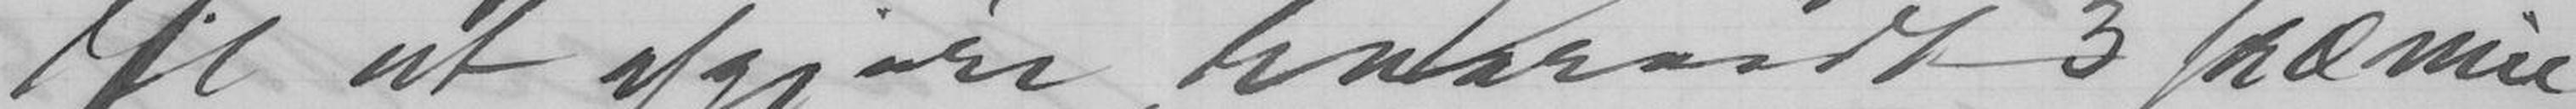til at afgjøre hvorvidt 3 præmie-
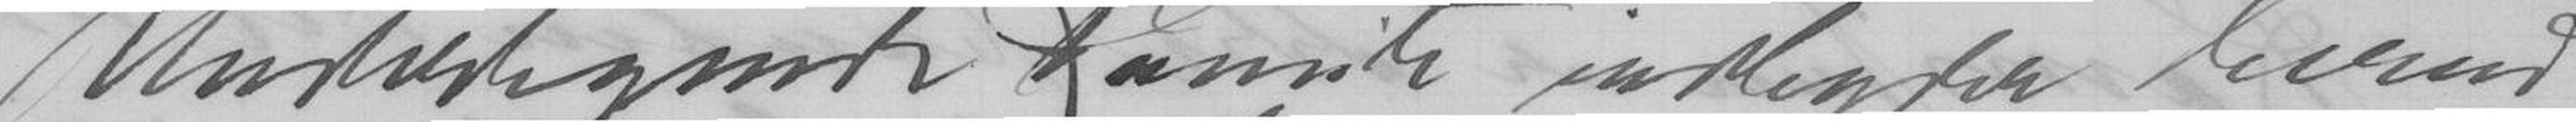Undertegnede Komite indbyder hermed
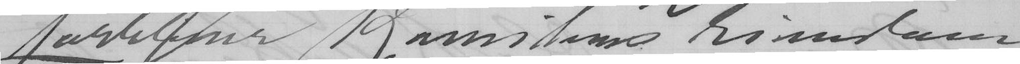forbliver Komiteens Eiendom
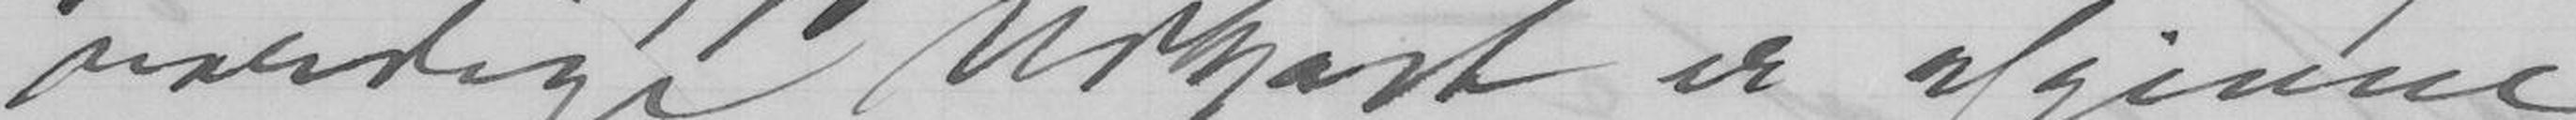værdige Udkast er afgivne
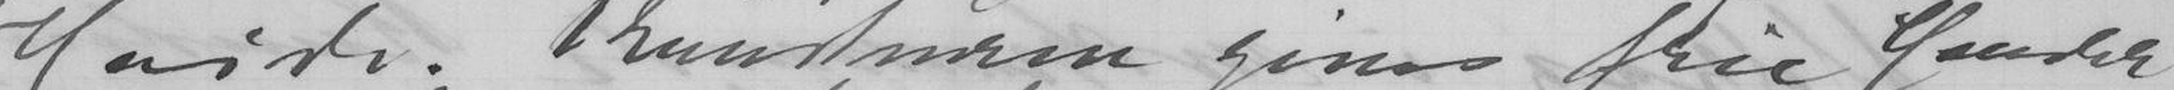Høide. Kunstnerne gives frie Hænder
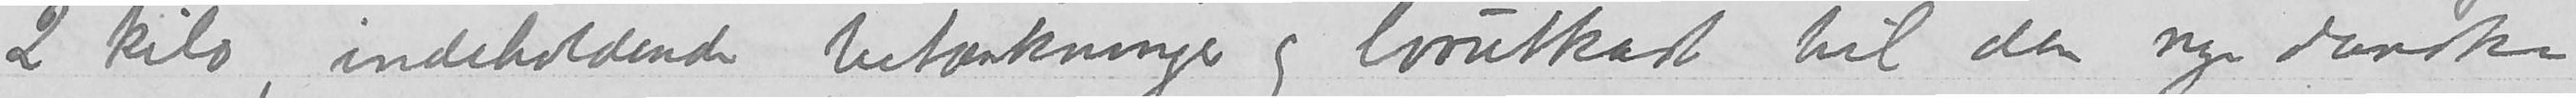2 kilo, indeholdende betænkninger og lovutkast til den nye danske
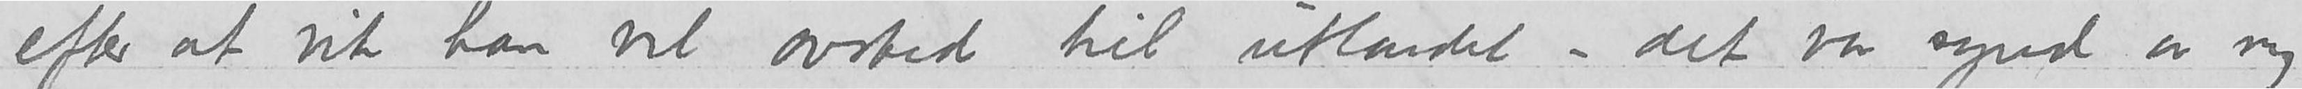efter at vite han vil av sted til utlandet – det var synd av mig
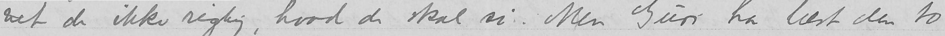vet de ikke rigtig, hvad de skal si.  Men Guri har læst den to
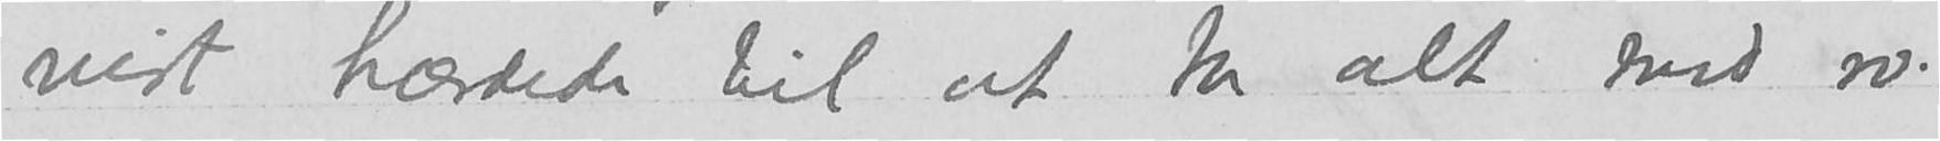vist <hærdede> til at ta alt med ro.
